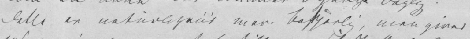Dette er naturligviis mere besparlig, men giver
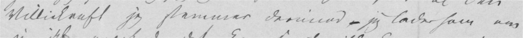Villiekraft jeg stemmer derimod – jeg lader som om
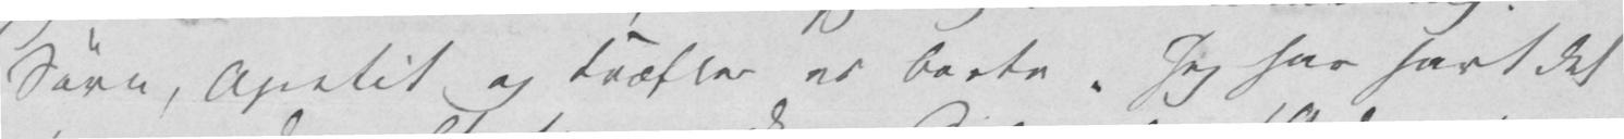Søvn, Apetit og Kræfter er borte. Jeg har havt det
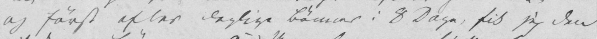og først efter daglige Bønner i 8 Dage, fik jeg den
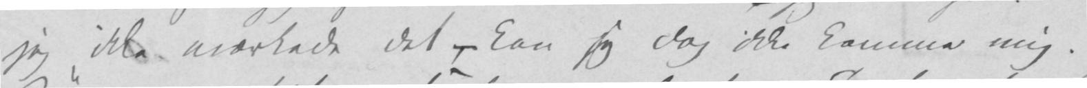jeg ikke mærkede det – kan jeg dog ikke komme mig.
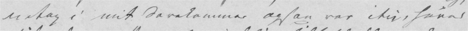netop i mit Sovekammer ogsaa var itu, hører
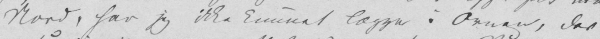Nord, har jeg ikke kunnet lægge i Ovnen, der
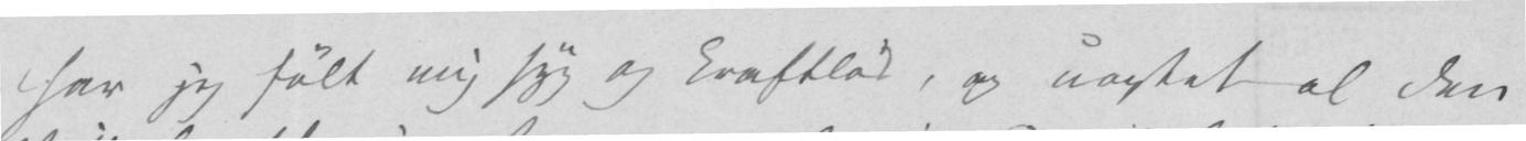har jeg følt mig syg og kraftløs, og uagtet al den
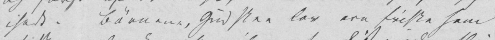isadt. Børnene, Gud skee lov ere friske som
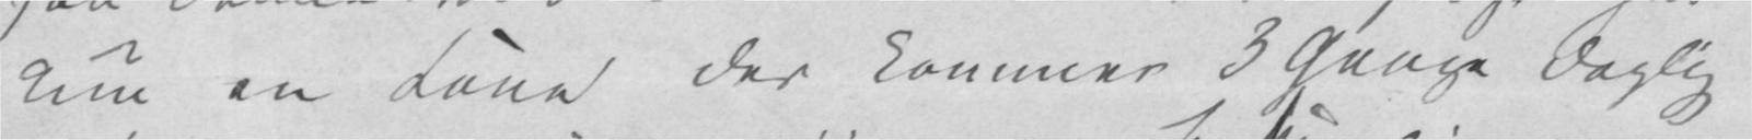kun en Kone der kommer 3 Gange daglig.
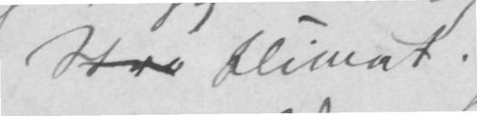Str Klimat.
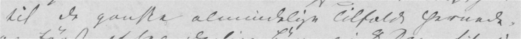til de ganske almindelige Tilfælde hernede,
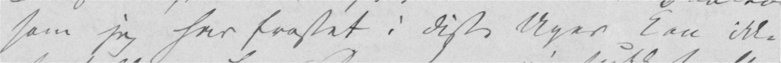som jeg har frosset i disse Uger kan ikke
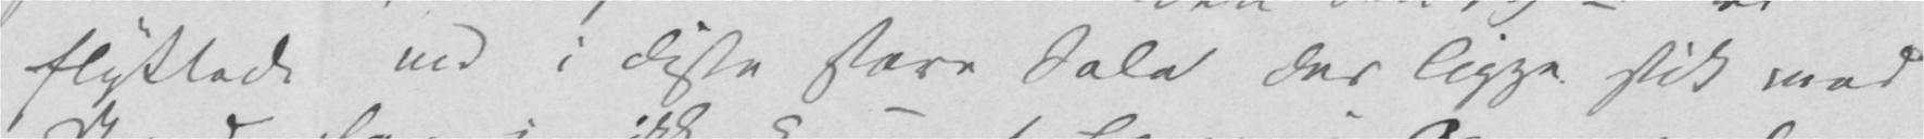flyttede ind i disse store Sale der ligge stik mod
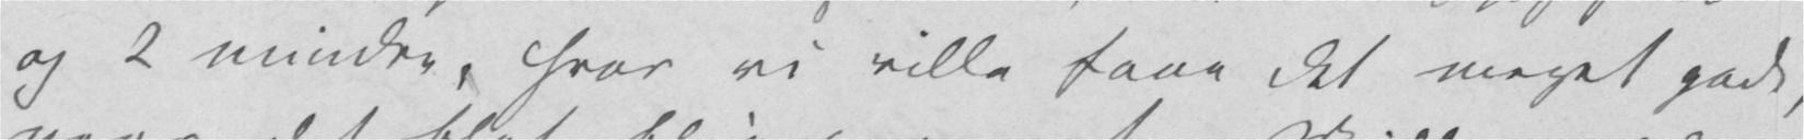og 2 mindre, hvor vi ville faae det meget godt,
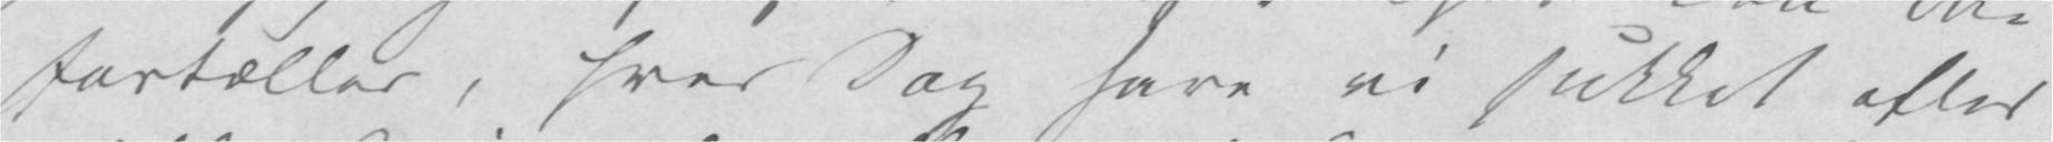fortælles, hver Dag have vi sukket efter
